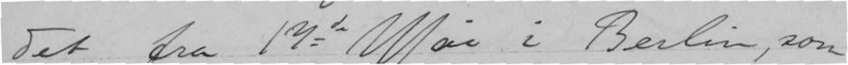det fra 17de Mai i Berlin, som
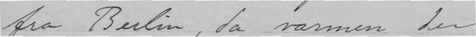fra Berlin, da varmen der
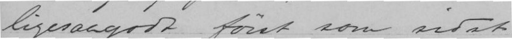ligesaagodt først som sidst
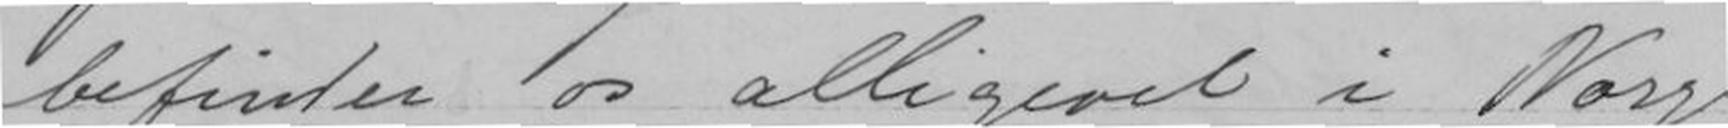befinder os alligevel i Norge,
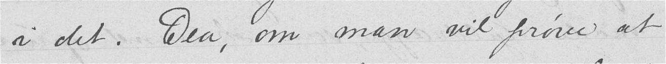i det. Dea, om man vil prøve at
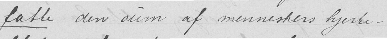fatte den sum af menneskers hjerte-
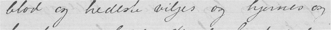blod og hedeste viljes og hjernes og
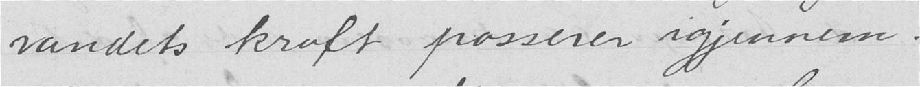vandets kraft passerer igjennem.
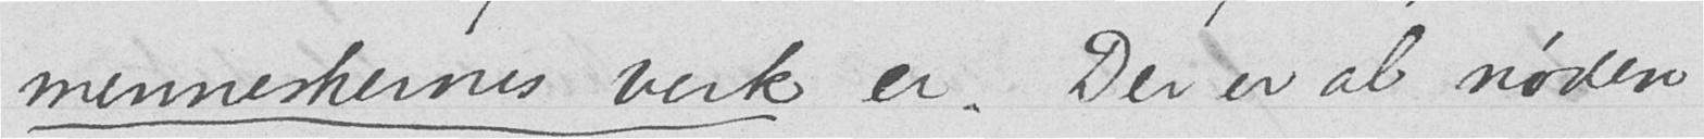menneskernes verk er. Der er al nøden
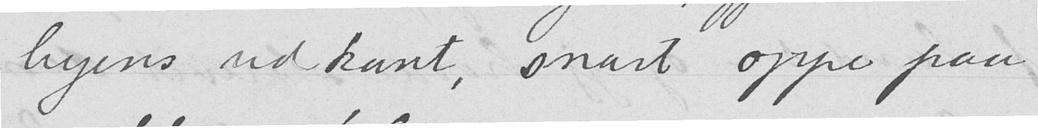byens udkant, snart oppe paa
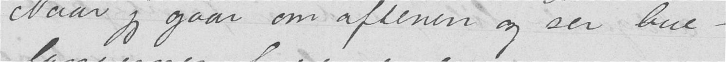Naar jeg gaar om aftenen og ser bue-
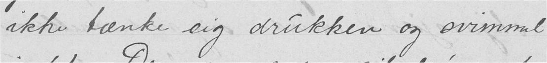ikke tænke sig drukken og svimmel
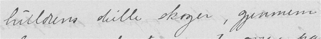huldrens stille skoger, gjennem
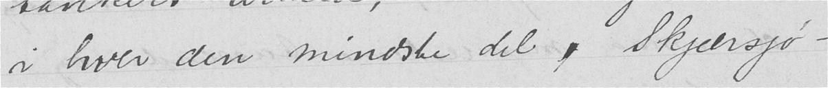i hver den mindste del  Skjærsjø-
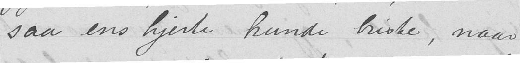saa ens hjerte kunde briste, naar
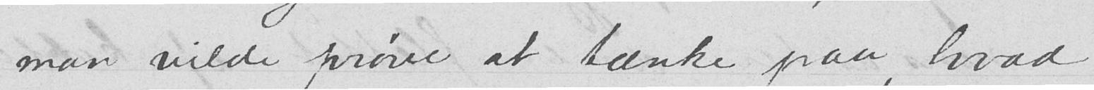man vilde prøve at tænke paa, hvad
